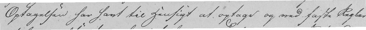Optagelsen har havt til Hensigt at optage og med faste Regler
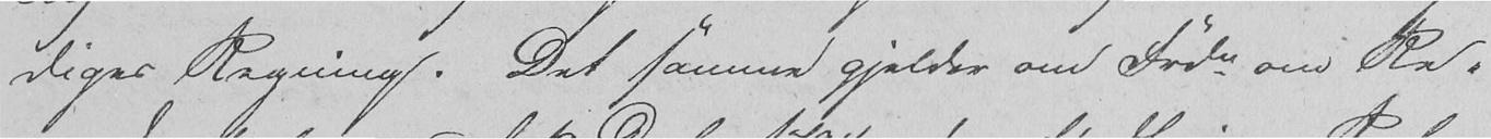diges Regning. Det samme gjelder om Frdn om Re-
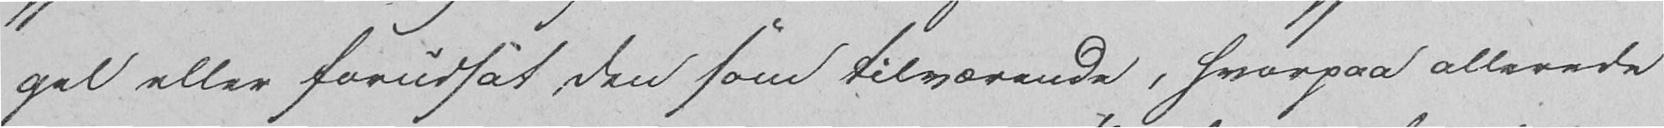gel eller forudsat den som tilværende, hvorpaa allerede
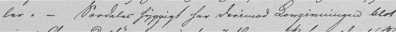ler. - Særdeles hyppigt har derimod Lovgivningen blot
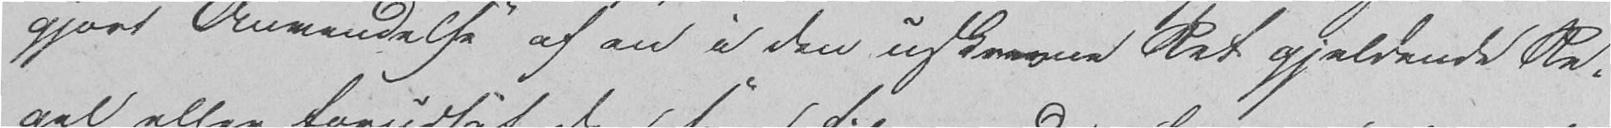gjort Anvendelse af en i den uskrevne Ret gjeldende Re-
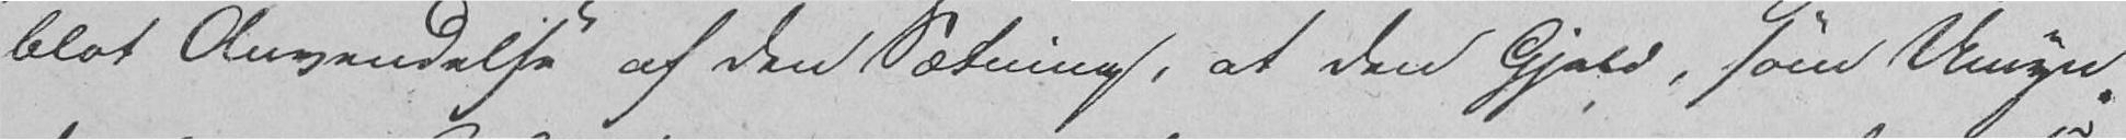blot Anvendelse af den Sætning, at den Gjeld, som Umyn-
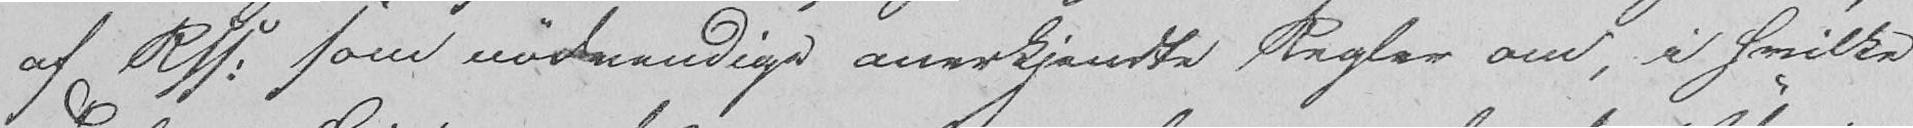af Res. som nødvendige anerkjendte Regler om, i hvilke
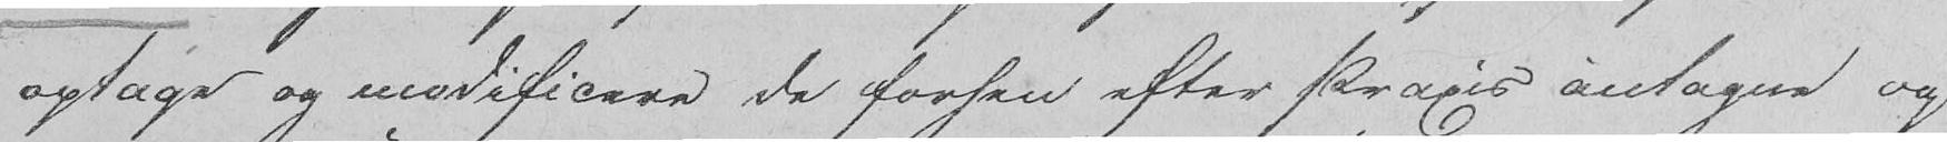optager og modificere de forhen efter Praxis antagne og
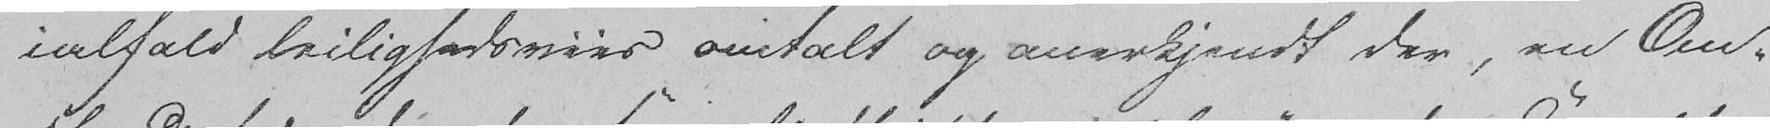ialfald leilighedsviis omtalt og anerkjendt der, en Om-
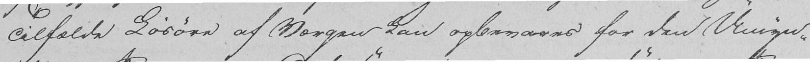Tilfælde Løsøre af Værgen kan opbevares for den Umyn-
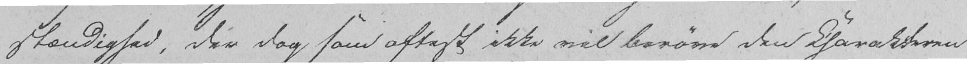stændighed, der dog som oftest ikke vil berøre den Kharakteren
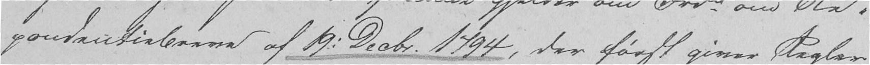pondentiaberne af 19. Decbr. 1794, der først giver Regler
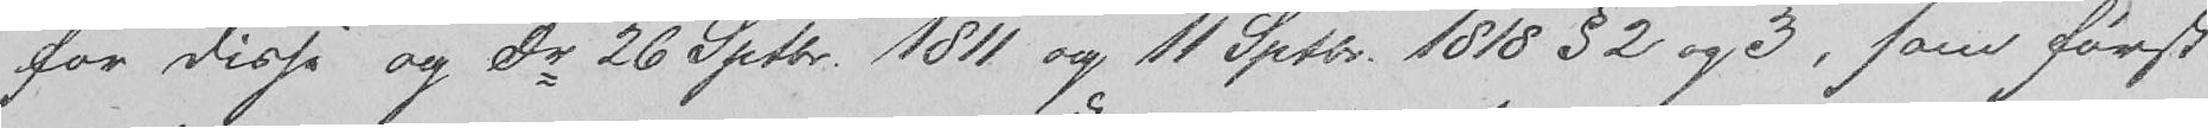for disse og Fr 26 Sptbr. 1811 og 11 Sptbr. 1818 § 2 og 3, som først
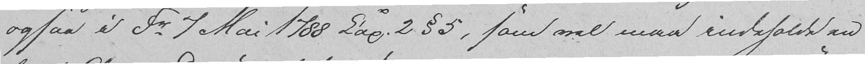ogsaa i Fr 7 Mai 1788 Kap. 2 § 5, som vel maa indeholde en
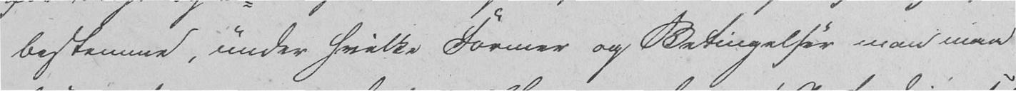bestemmer, under hvilke Former og Betingelser man maa
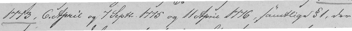1773, 6. April og 7 Septr. 1775 og 11 April 1776, samtlige § 1, der
